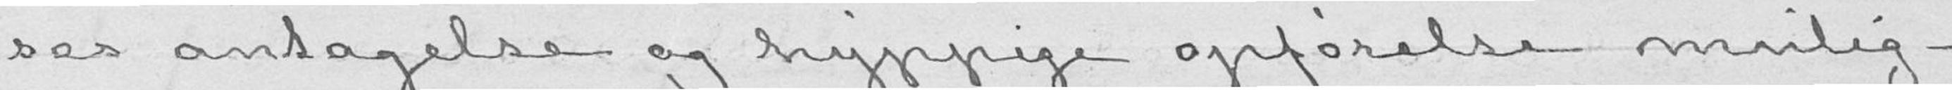ses antagelse og hyppige opførelse mulig-
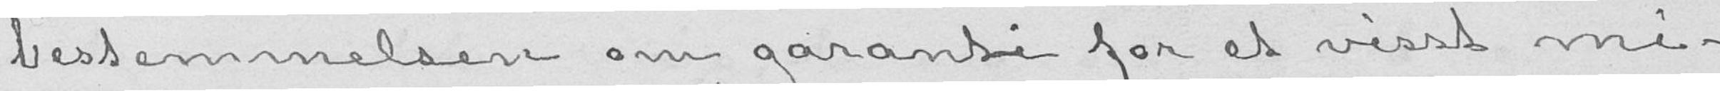bestemmelsen om garanti for et visst mi-
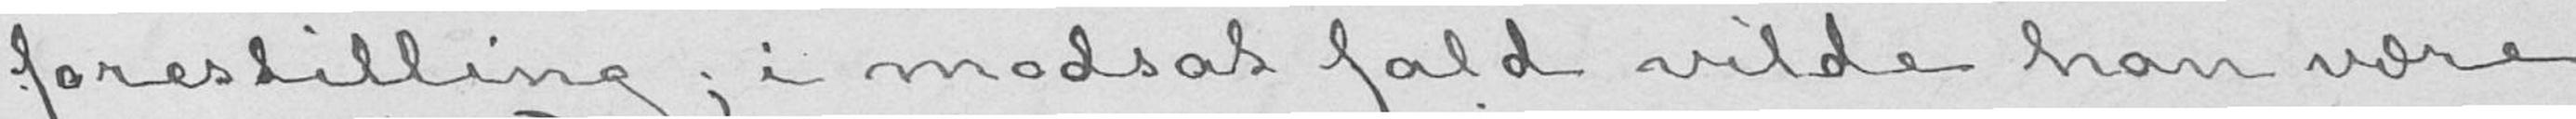forestilling; i modsat fald vilde han være
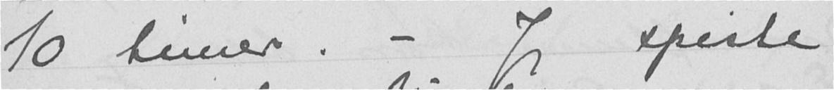10 timer. - Jeg spiste
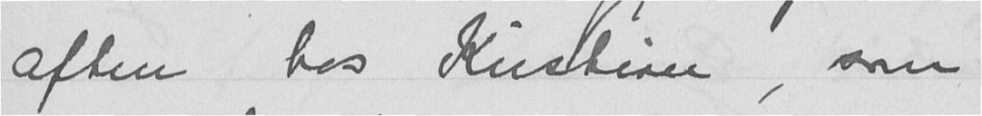aften hos Kristian, som
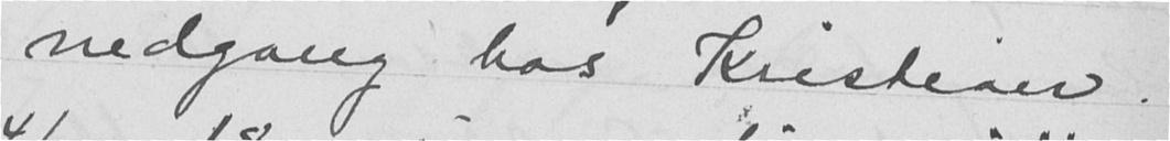nedgang hos Kristian.
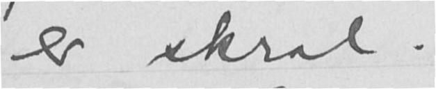er skral.
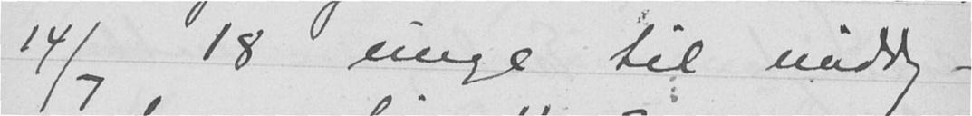14/7 18 unge til middag -
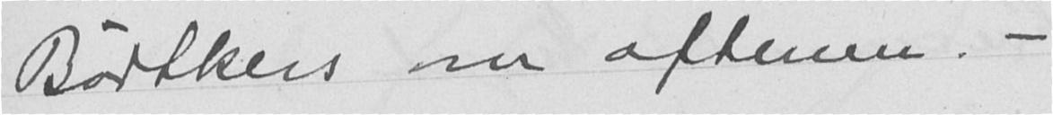Bødtkers om aftenen. -
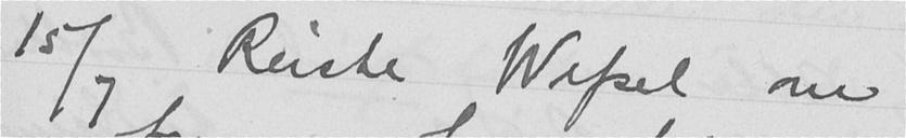15/7 Reiste Wessel om
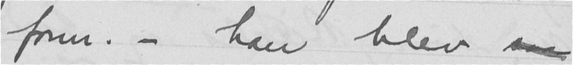form. - han blev saa
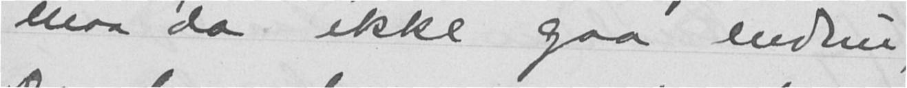maa da ikke gaa endnu,
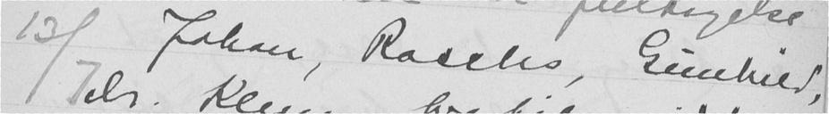13/7 Johan, Raschs, Gunhild,
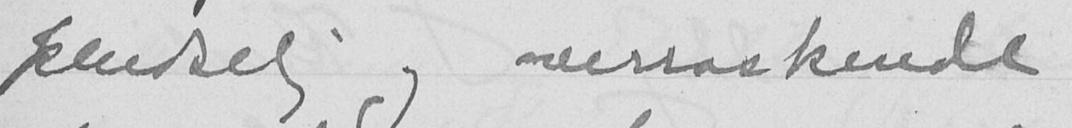pludselig og overraskende
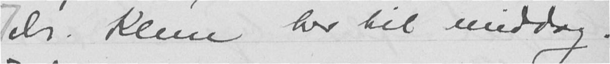dr. Klem her til middag.
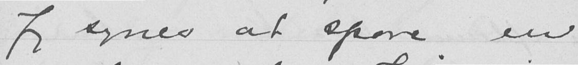Jeg synes at spore en
